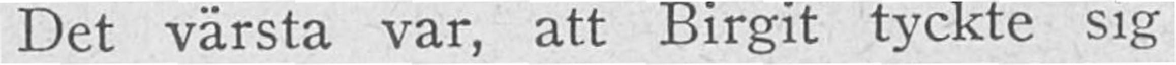Det värsta var, att Birgit tyckte sig
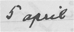5 april.
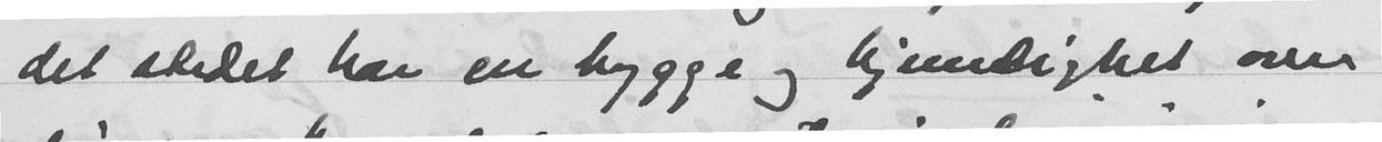det stedet har en hygge og hjemlighet over
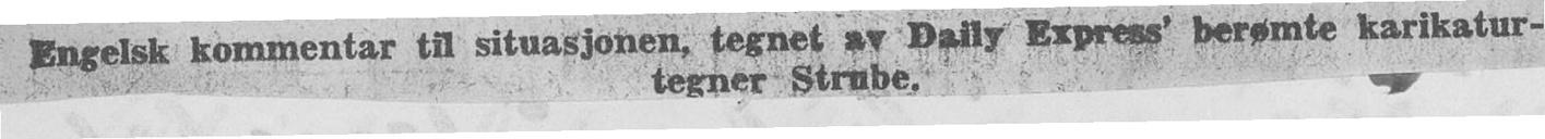Engelsk kommentar til situasjonen, tegnet av Daily Express' berømte karikatur-
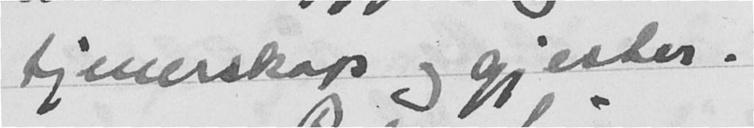tjenerskap og gjester.
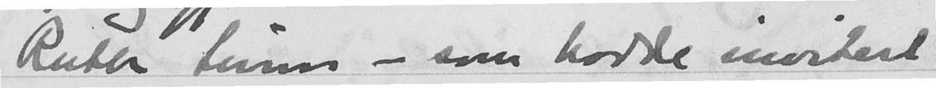Ruth Leum - som hadde invitert
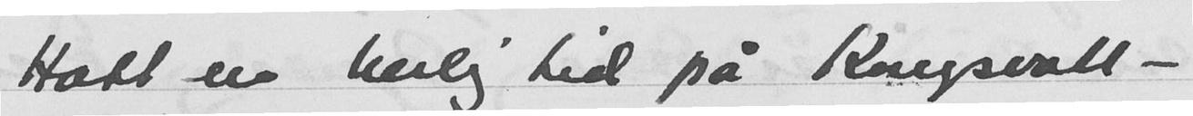Hatt en herlig tid på Kongsvoll -
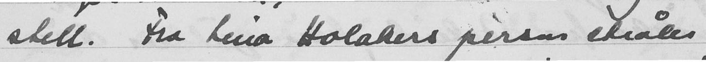stell. Fra Lina Holakers person stråler
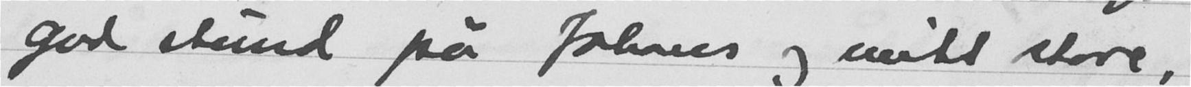god stund på Johans og mitt store,
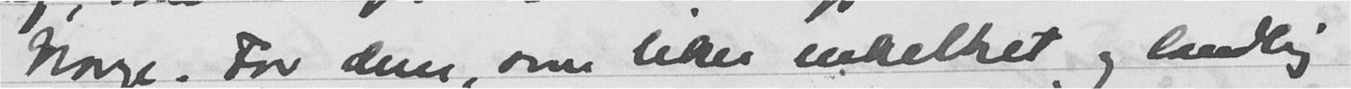Norge. For dem, som liker enkelhet og landlig
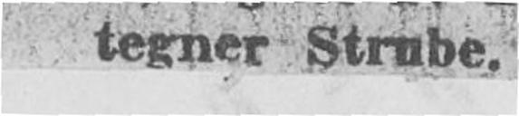tegner Strube.
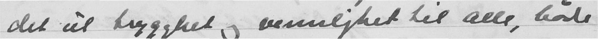det ut tryggtet og vennlighet til alle, både
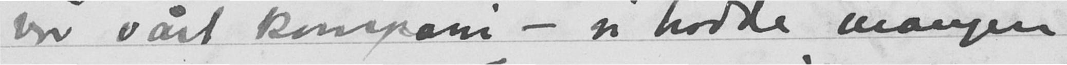var vårt kompani - jeg hadde mangen
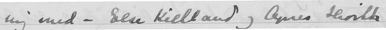mig med - Else Kielland og Agnes Hiorth
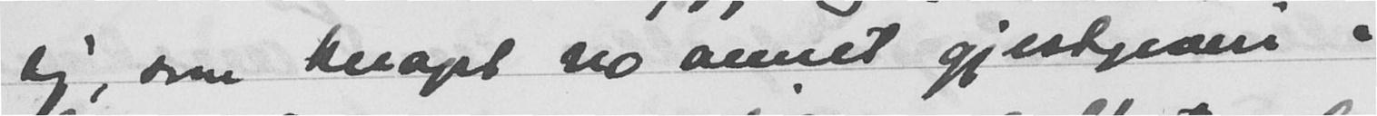sig, som knapt no annet gjestgiveri i
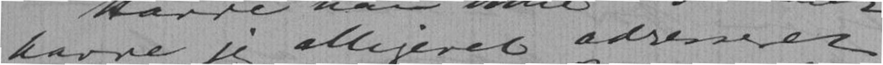havde jeg alligevel adresseret
